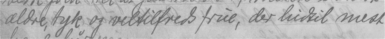ældre, tyk og veltilfreds frue, der hidtil mest
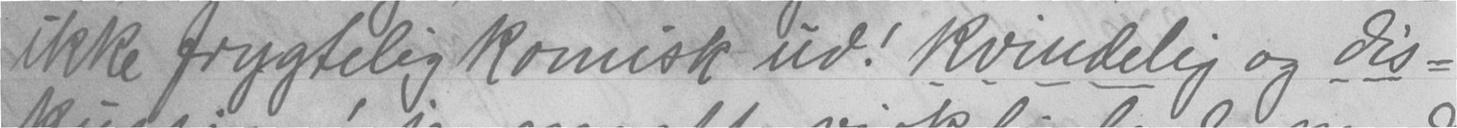ikke frygtelig komisk ud! kvindelig og dis-
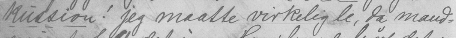kussion! jeg maatte virkelig le, da mand-
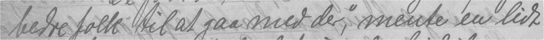bedre folk til at gaa med der," mente en lidt
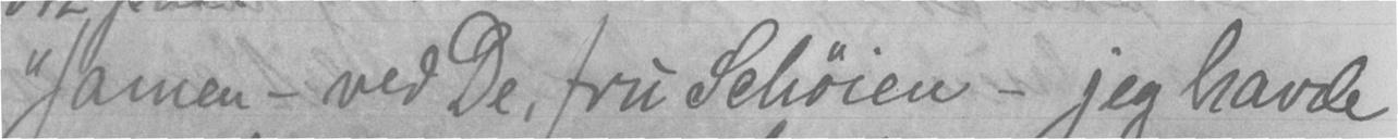"Jamen - ved De, fru Schøien - jeg havde
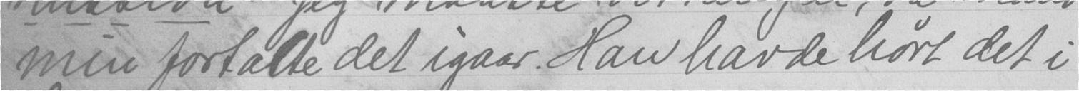min fortalte det igaar. Han havde hørt det i
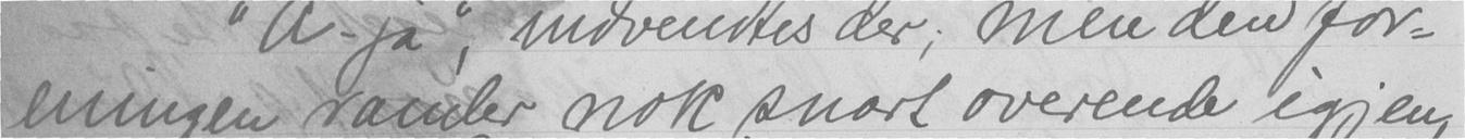eningen ramler nok snart overende igjen,
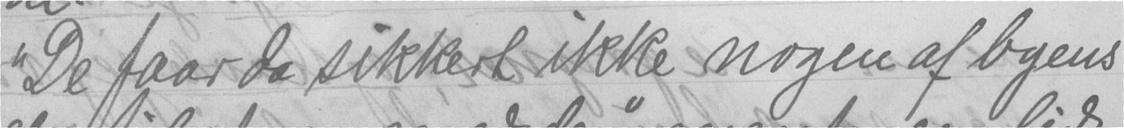"De faar da sikkert ikke nogen af byens
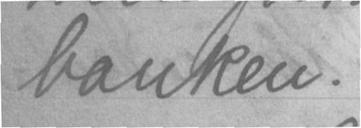banken."
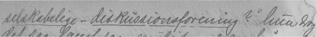selskabelige - diskussionsforening?" hun drog
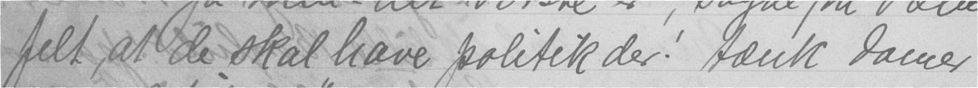felt, at de skal have politik der! tænk damer
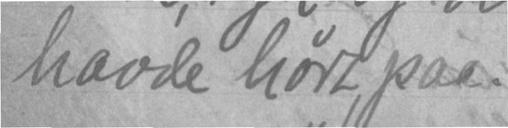havde hørt paa.
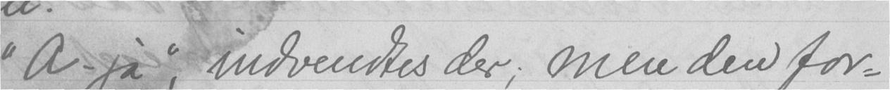"A-ja", indvendtes der; men den for-
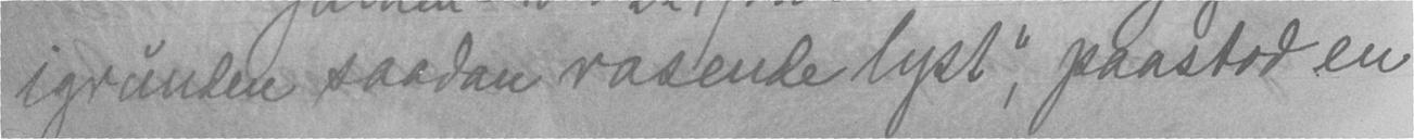igrunden saadan rasende lyst," paastod en
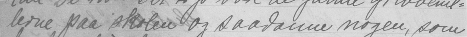lerne paa skolen og saadanne nogen, som
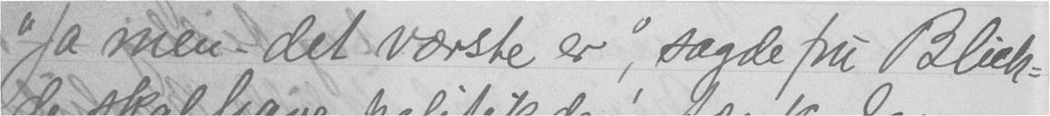"Ja men - det værste er", sagde fru Blick-
